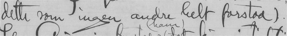dette som ingen andre helt forstod).
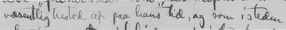væsentlig bestod af paa hans tid, og som isteden
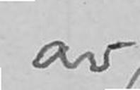av
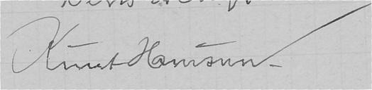Knut Hamsun.
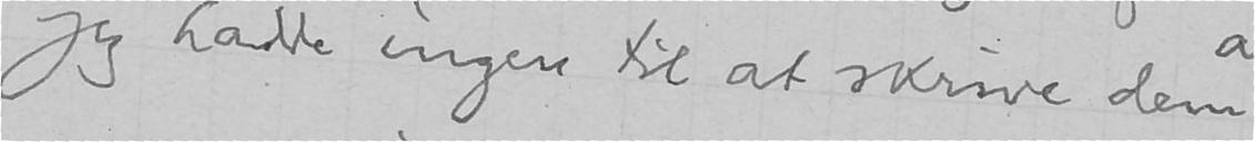jeg hadde ingen til at skrive dem
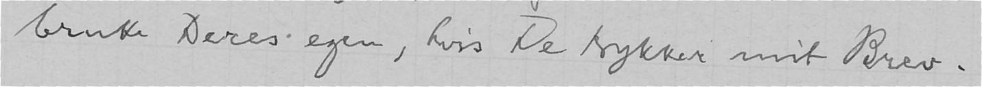bruke Deres egen, hvis De trykker mit Brev.
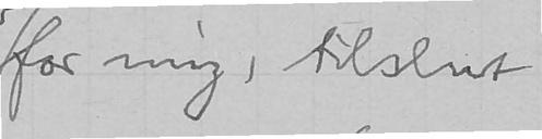for mig, tilslut
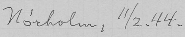Nørholm, 11/2. 44.
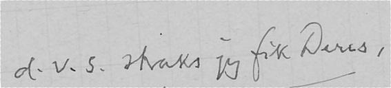d.v.s. straks jeg fik Deres,
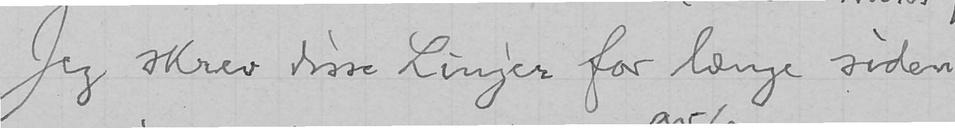Jeg skrev disse Linjer for længe siden,
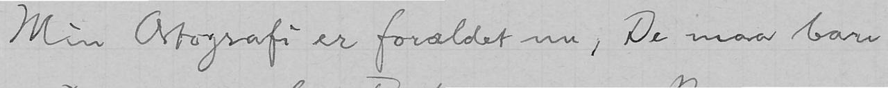Min Ortografi er forældet nu, De maa bare
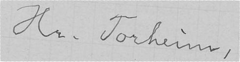Hr. Torheim,
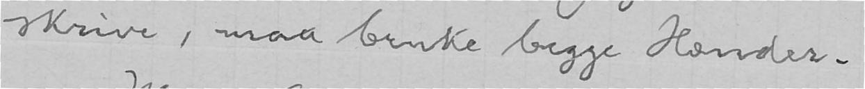skrive, maa bruke begge Hænder.
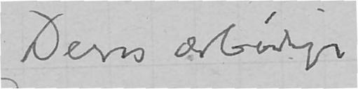Deres ærbødige
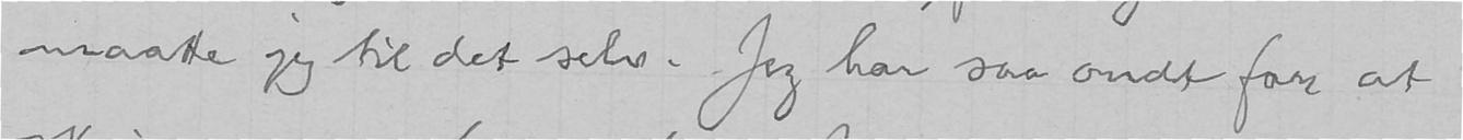maatte jeg til det selv. Jeg har saa ondt for at
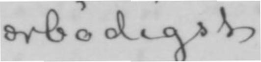ærbødigst
